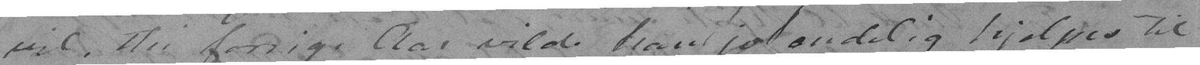vil, thi forrige Aar vilde han jo endelig hjelpes til
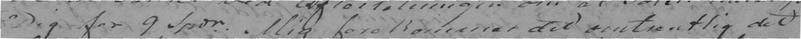Dig for 9 Spdo. Mig forekommer det omtrentlig det
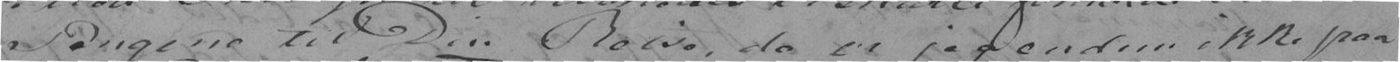Pengene til Din Reise, da er jeg endnu ikke paa
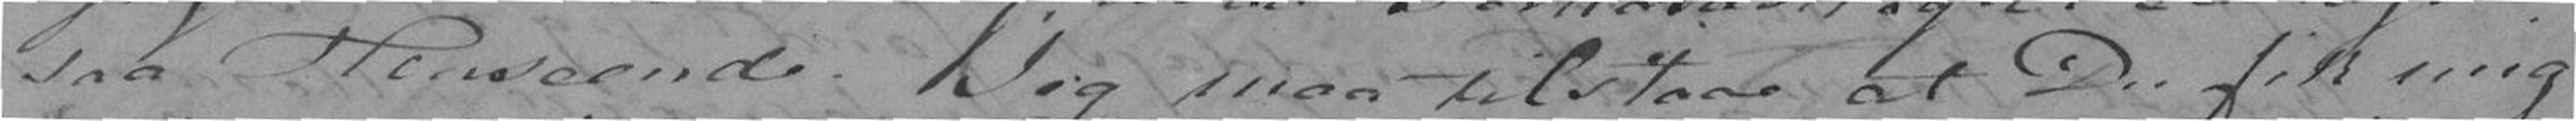saa Henseende. Jeg maa tilstaae at Du fik mig
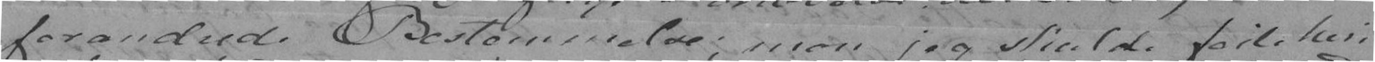forandrede Bestemmelse; mon jeg skulde feile heri?
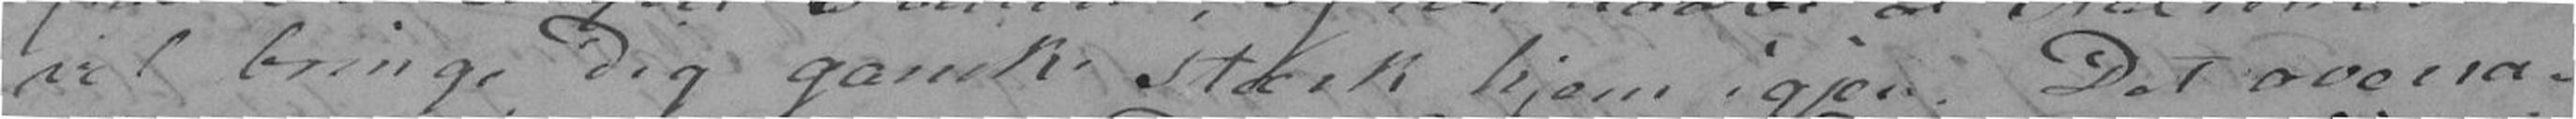vil bringe Dig ganske stærk hjem igjen. Det overra-
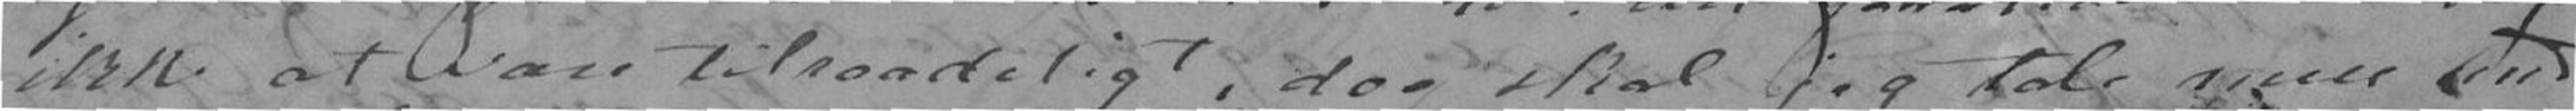ikke at være tilraadeligt, dog skal jeg tale mere med
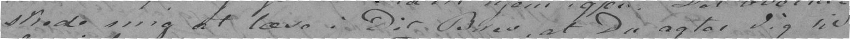skede mig at læse i Dit Brev, at Du agter Dig til
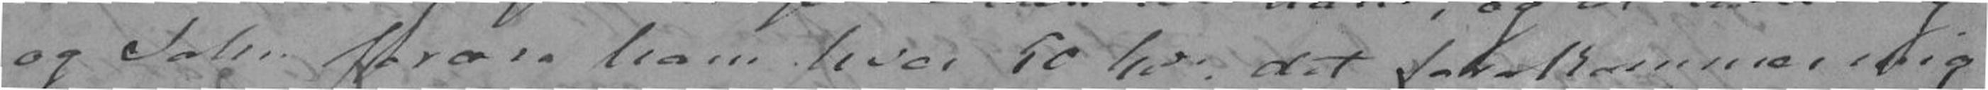og John forære ham hver 50Spdo, det forekommer mig
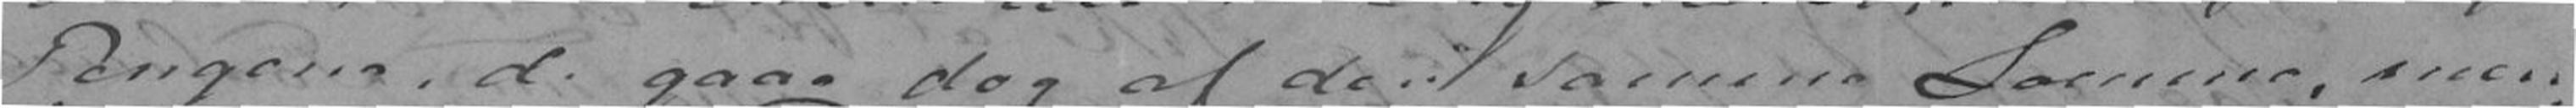Pengene, de gaae dog af den samme Lomme, men
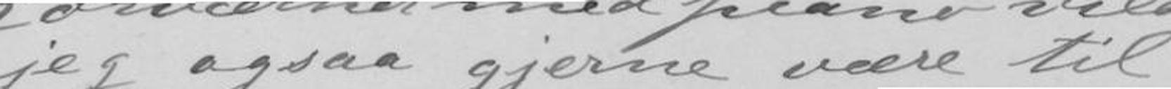jeg ogsaa gjerne være til
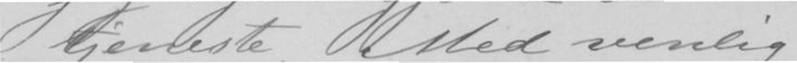tjeneste. Med venlig
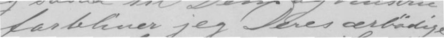forbliver jeg Deres ærbødige
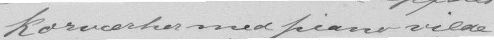korværker med piano vilde
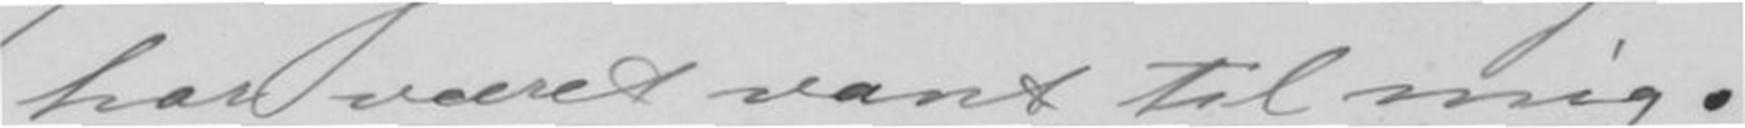har været vant til mig.
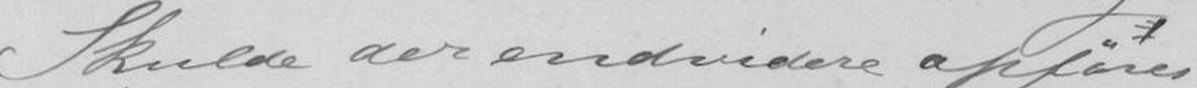Skulde der endvidere opføres
